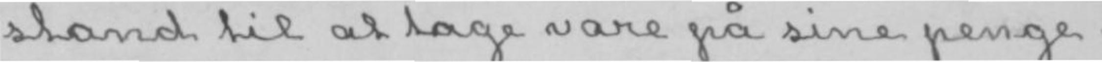stand til at tage vare på sine penge.
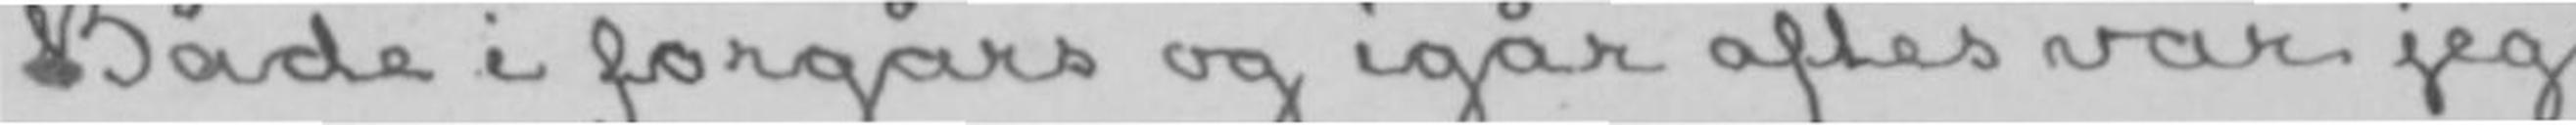Både i forgårs og igår aftes var jeg
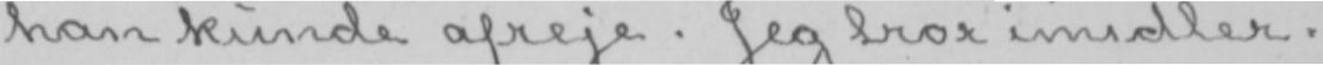han kunde afreje. Jeg tror imidler-
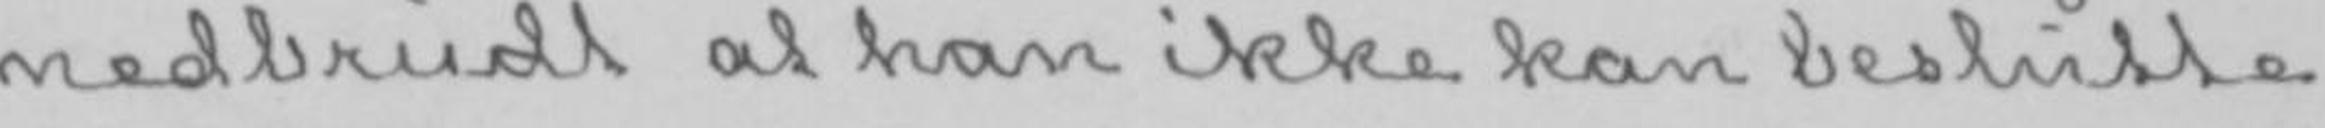nedbrudt at han ikke kan beslutte
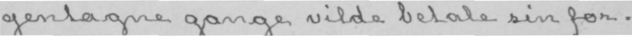gentagne gange vilde betale sin for-
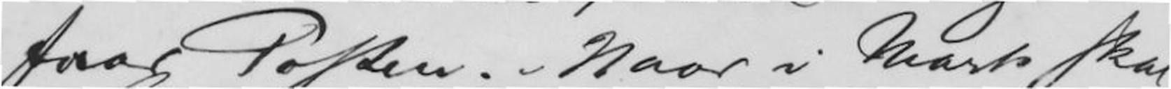faar Posten. - Naar i Marts skal
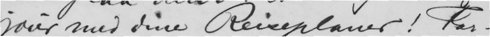jour med dine Reiseplaner! Far-
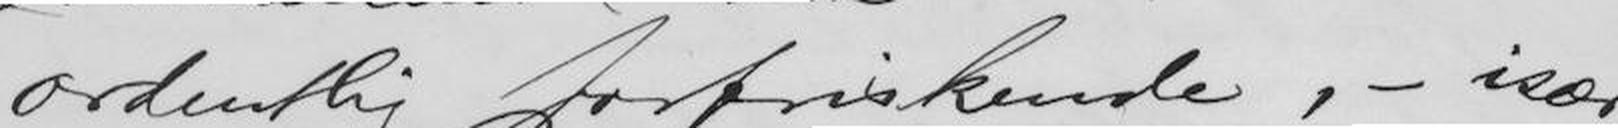ordentlig forfriskende, - især
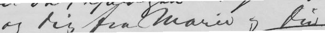og dig fra Marie og Din
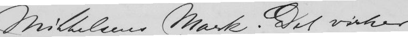Mikkelsens Mark. Det virker
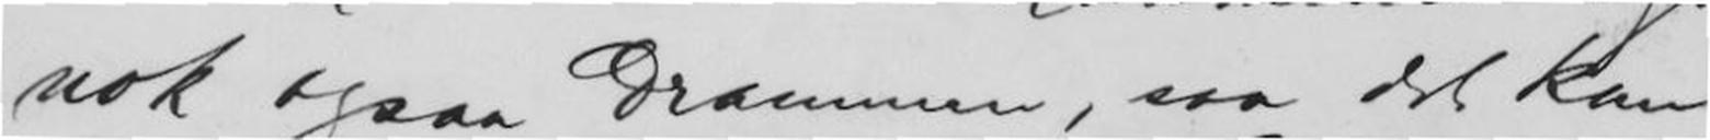nok ogsaa Drammen, saa det kan
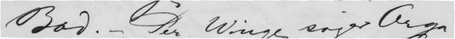Bad. Per Winge søger Orga-
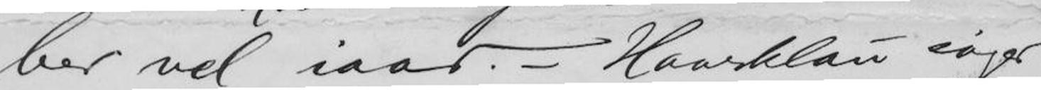ber vel iaar. - Haarklou søger
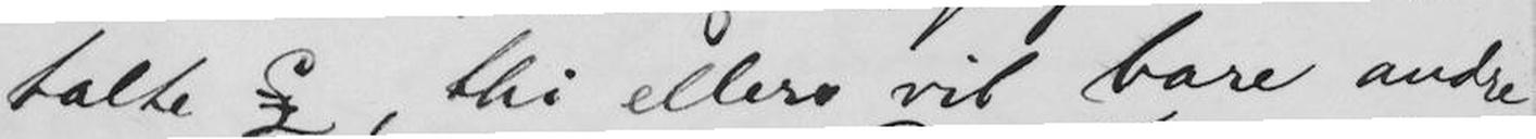talte £, thi ellers vil bare andre
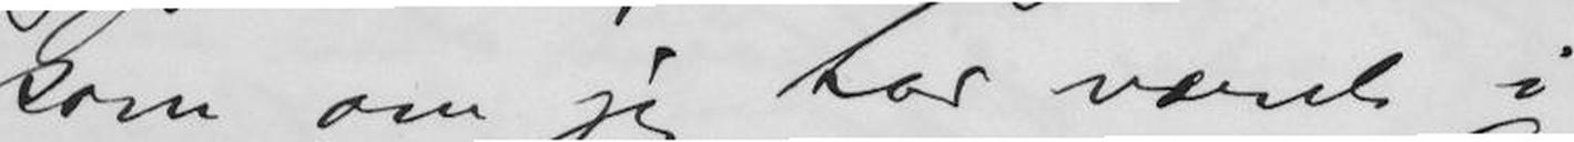som om jeg har været i
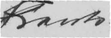Frants.
